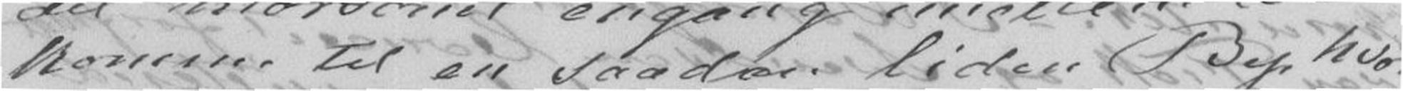komme til en saadan liden By hvor
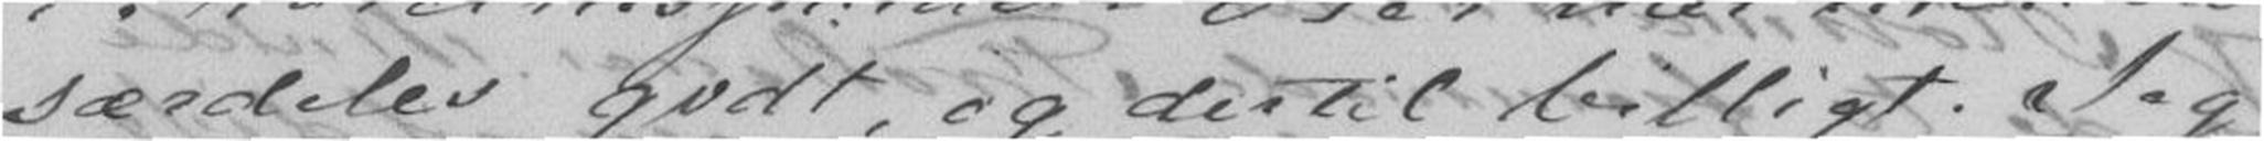særdeles godt, og dertil billigt. Jeg
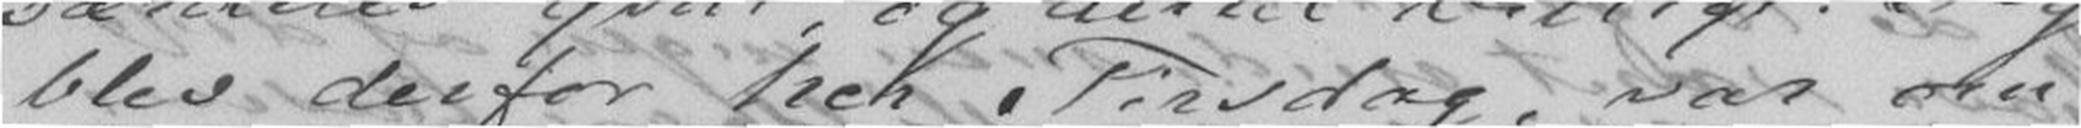blev derfor her Tirsdag, var om
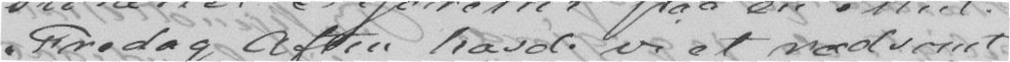Fredag Aften havde vi et rædsomt
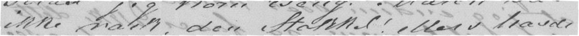ikke rask, den Stakkel! ellers havde
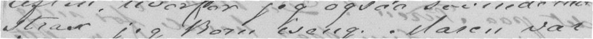strax jeg kom iseng. Maren var
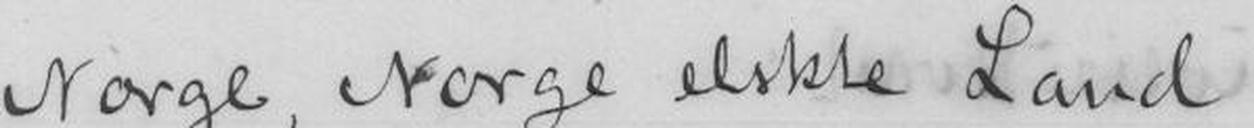Norge, Norge elskte Land
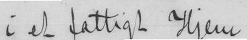i et fattigt Hjem
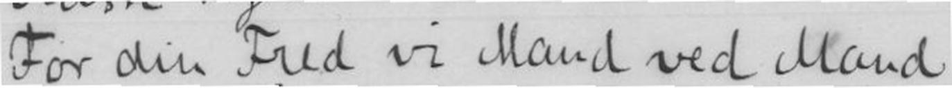For din Fred vi Mand ved Mand
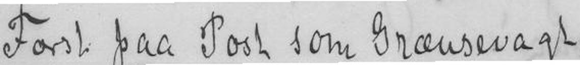Først paa Post som Grænsevagt
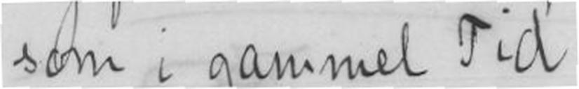som i gammel Tid
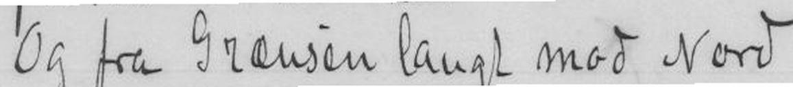Og fra Grændsen langt mod Nord
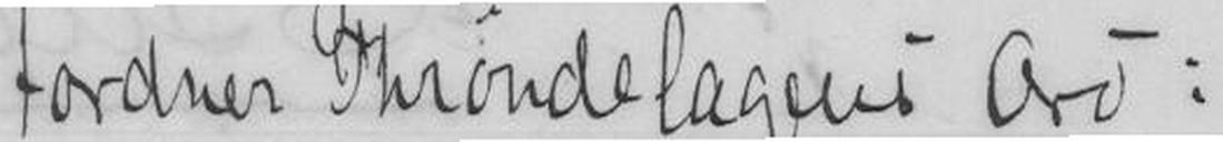:/:tordner Trøndelagens Ord:
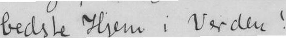bedste Hjem i Verden!
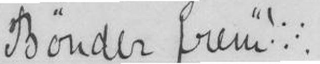Bønder frem!:/:
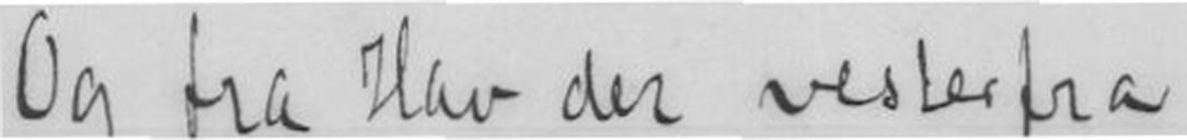Og fra Hav der vesterfra
